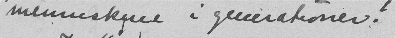menneskene i generationer!
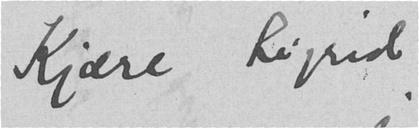Kjære Sigrid
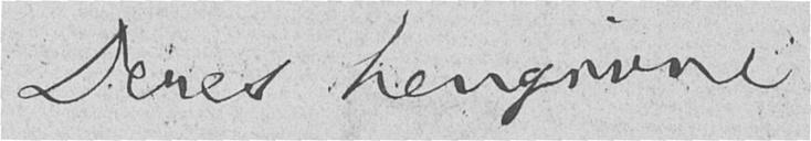Deres hengivne
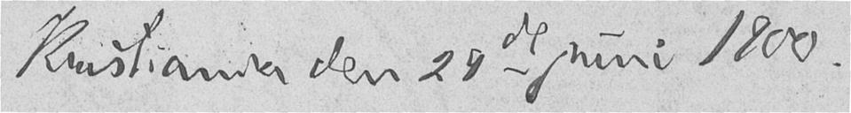Kristiania den 29de juni 1900.
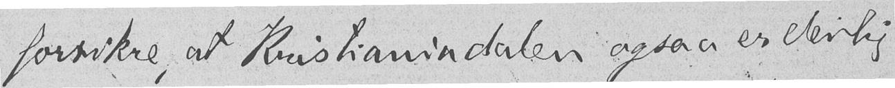forsikre, at Kristianiadalen ogsaa er deilig
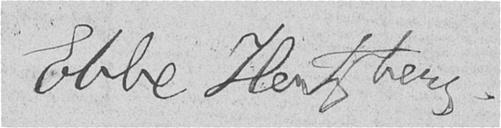Ebbe Hertzberg.
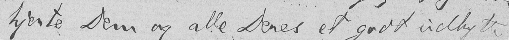hjerte Dem og alle Deres et godt udbytte
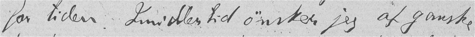for tiden. Imidlertid ønsker jeg af ganske
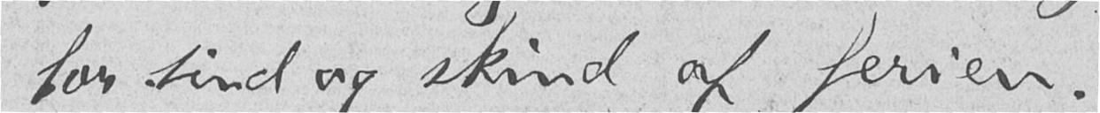for sind og skind af ferien.
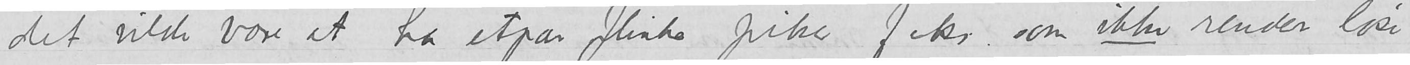det vilde vore at ha etpar flinke piker f eks. som ikke render løse
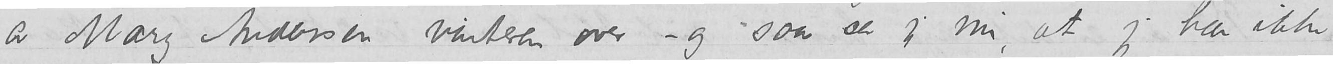av Mary Andersen vinteren over – og saa ser jeg nu, at jeg har ikke
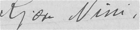Kjære Nini,
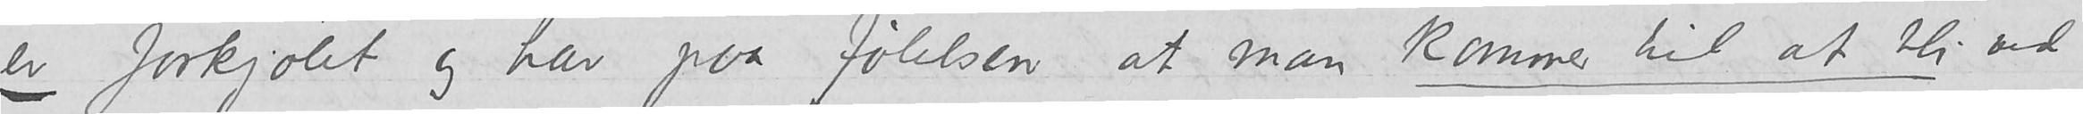er forkjølet og har paa følelsen at man kommer til at bli ved
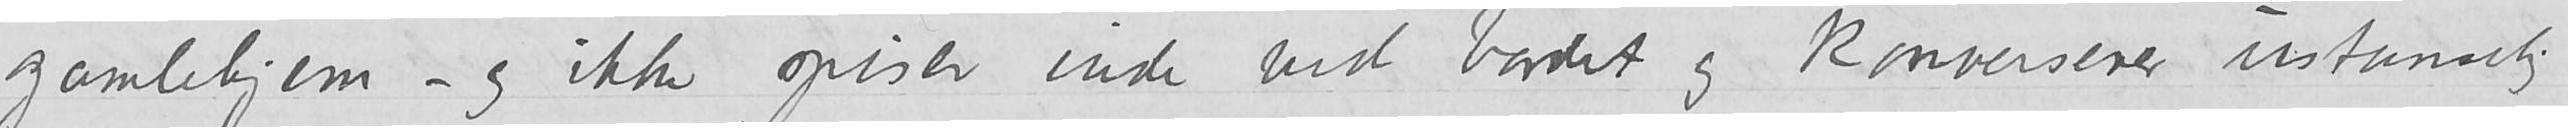gamlehjem – og ikke spiser inde ved bordet og konverserer ustanselig
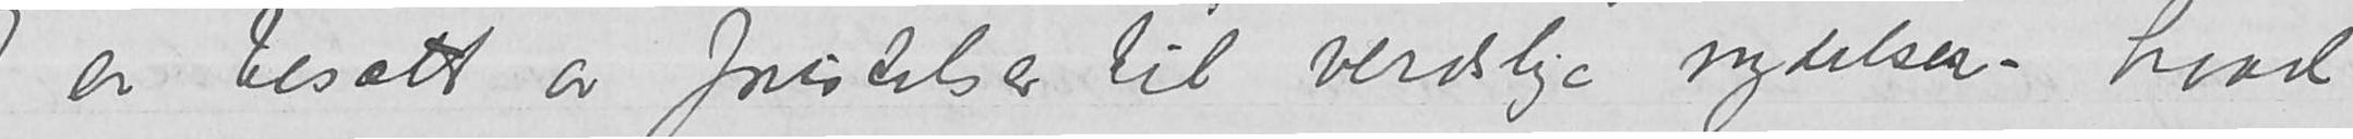Jeg er besatt av fristelser til verdslige nydelser – hvad
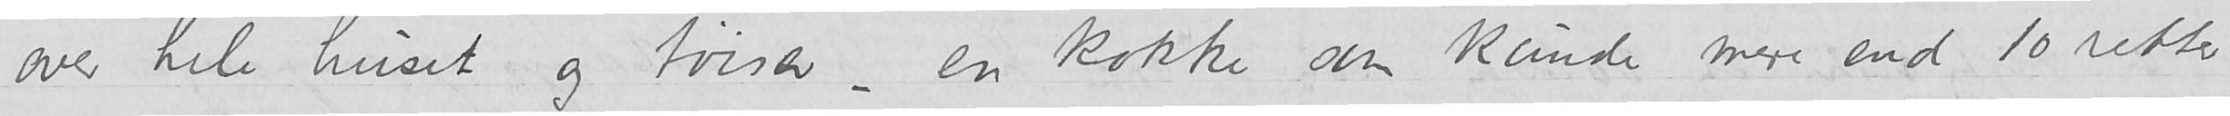over hele huset og tøiser – en kokke som kunde mere end 10 retter
